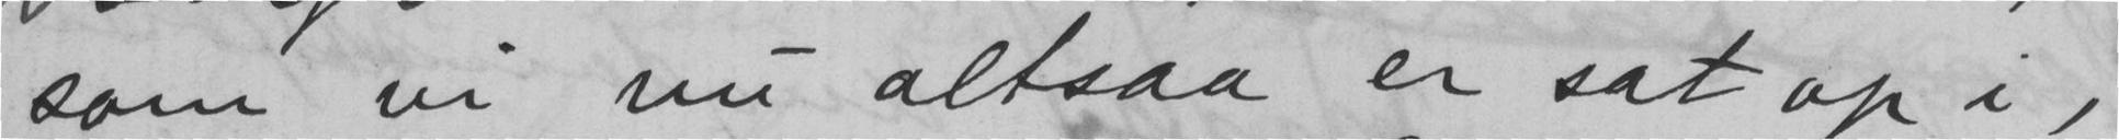som vi nu altsaa er sat op i,
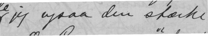jeg ogsaa den stærke
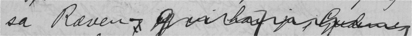sa Ræven
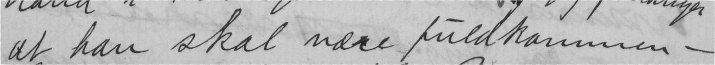at han skal være fuldkommen -
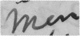Men
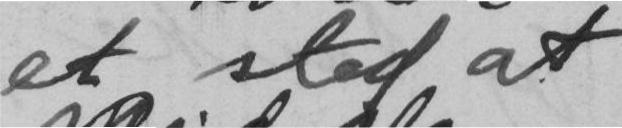et sted at
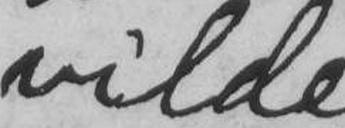vilde
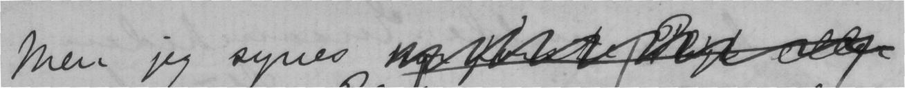Men jeg synes
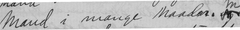Mand i mange Maader.
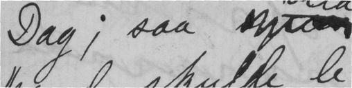Dag; saa
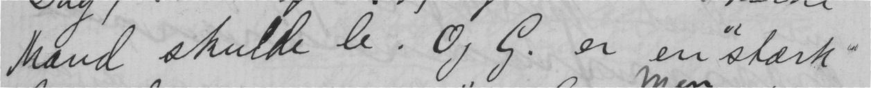Mand skulde le. Og G. er en "stærk"
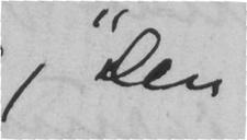, "den
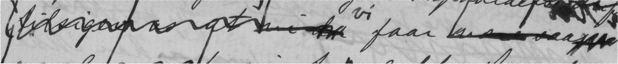faar
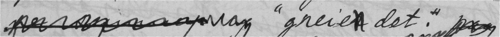"greie det".
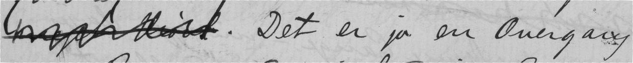. Det er jo en Overgang
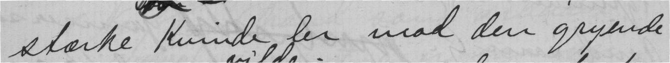stærke Kvinde ler mod den gryende
